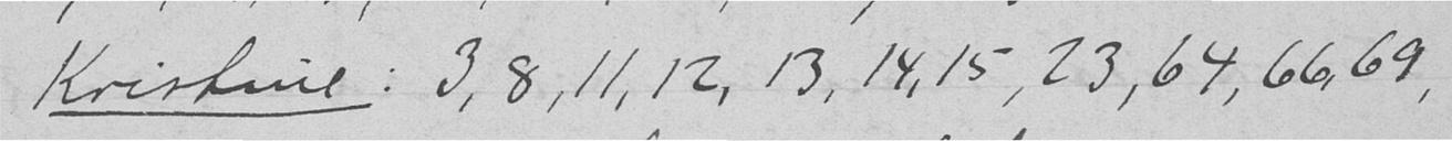Kristine: 3, 8, 11, 12, 13, 14, 15, 23, 64, 66, 69,
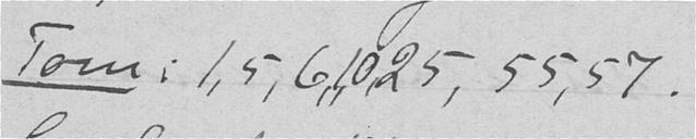Tom: 1, 5, 6, 10, 25, 55, 57.
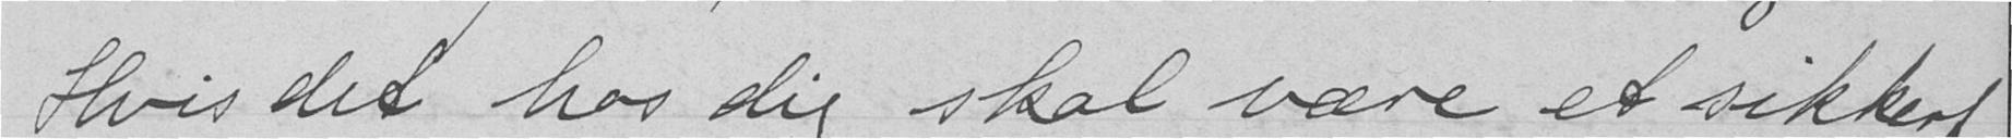Hvis det hos dig skal være et sikkert
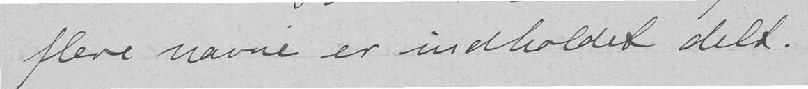flere navne er indholdet delt.
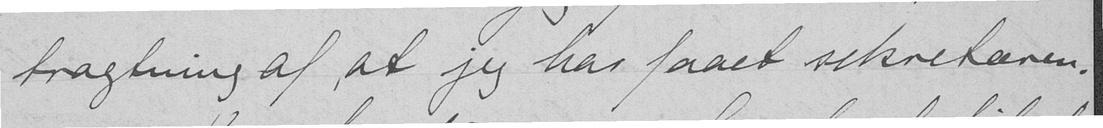tragtning af, at jeg har faaet sekretæren.
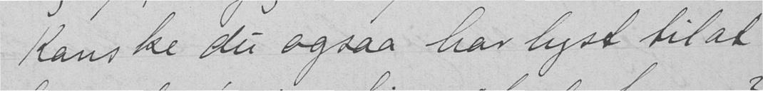Kanske du ogsaa har lyst til at
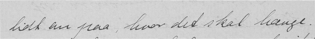lidt an paa, hvor det skal hænge.
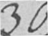30
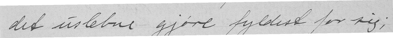det uslebne gjøre fyldest for sig;
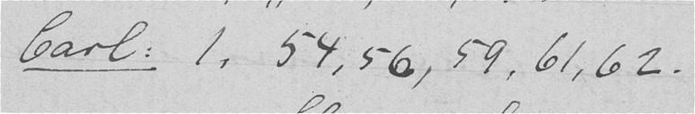Carl: 1, 54, 56, 59, 61, 62.
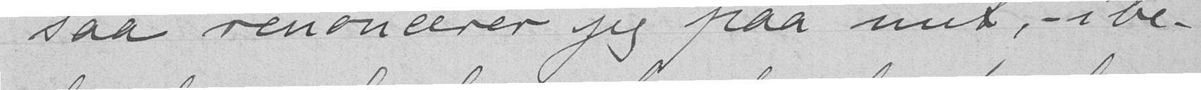renoncerer jeg paa mit, - i be-
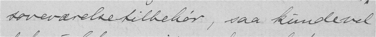soveværelsetilbehør, saa kunde vel
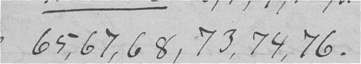65, 67, 68, 73, 74, 76.
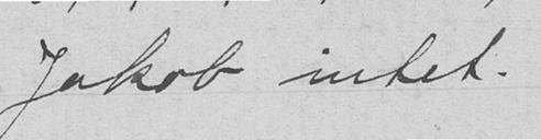Jakob intet.
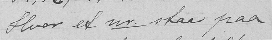Hvor et nr. staar paa
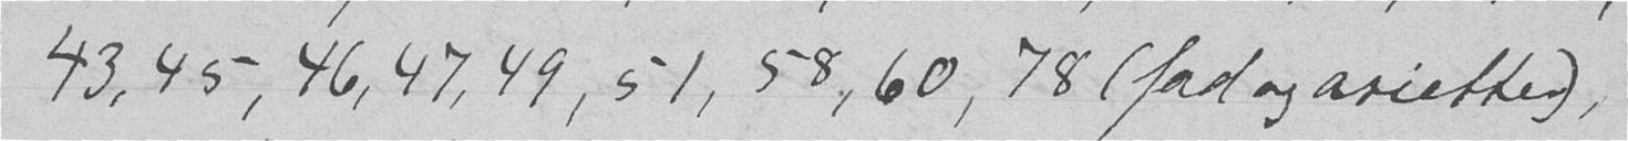43, 45, 46, 47, 49, 51, 58, 60, 78 (fad og asietter),
